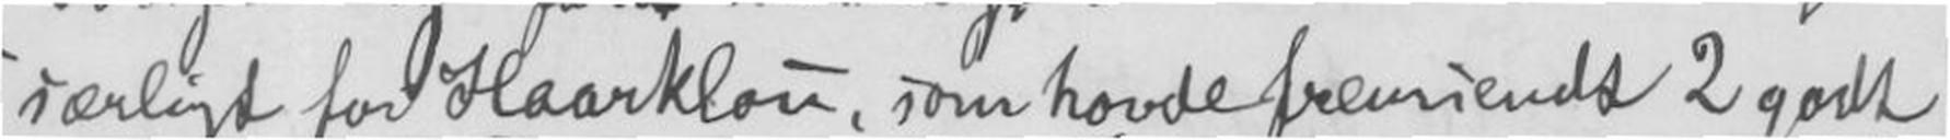særligt for Haarklou, som havde fremsendt 2 godt
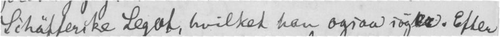Schäfferske Legat, hvilket han ogsaa søgte. Efter
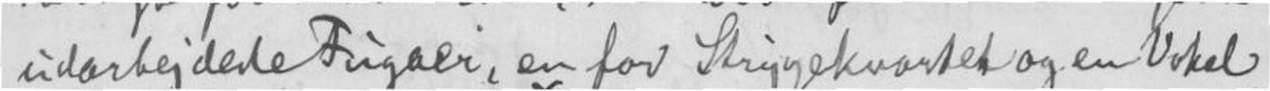udarbejdede Fugaer, en for Strygekvartet og en Vokal-
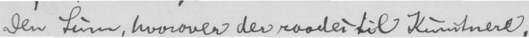Den Sum, hvorover der raades Kunstnere,
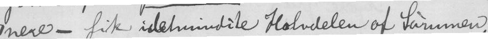nere - fik idetminste Halvdelen af Summen,
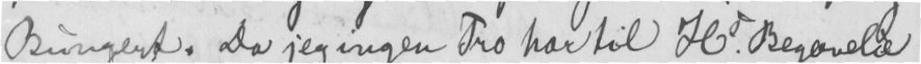Bungert. Da jeg ingen Tro har til Hs. Begavelse
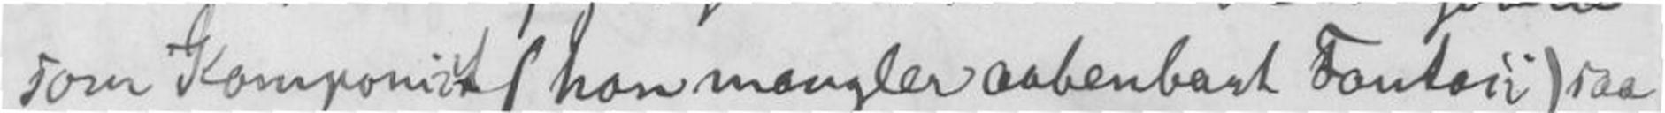som Komponist (han mangler aabenhed Fantasi) saa
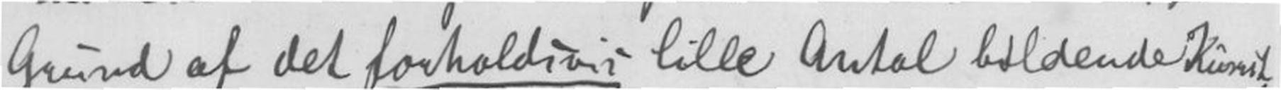Grund af der forholdsvis lille Antal bildende Kunst-
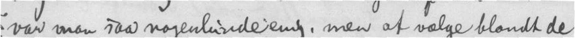var man saa nogenlunde enig, men at vælge blandt de
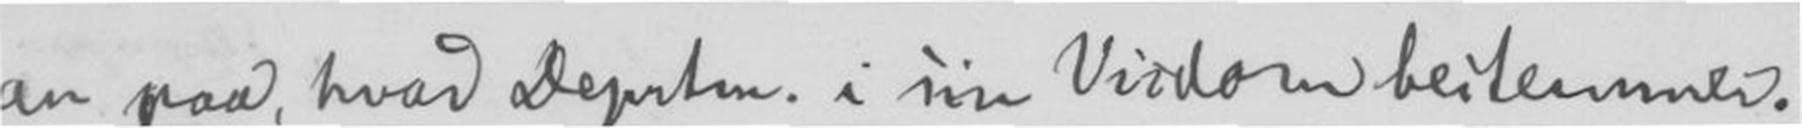an paa, hvad Deprtm. i sin Visdom bestemmer.
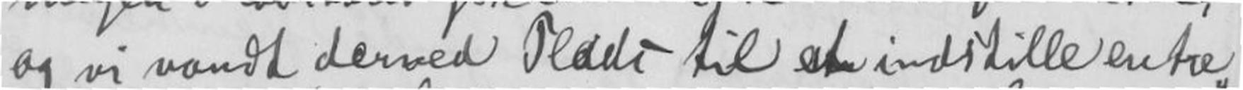og vi vandt derved Plads til at indstlle en tre-
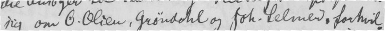sig om O. Olsen, Grøndahl og Joh. Selmer, for hvil-
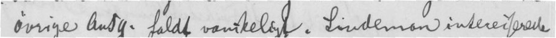øvrige Ansg. faldt vanskeligt. Lindeman interesserede
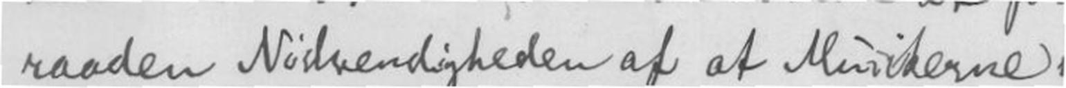raaden Nødvendigheden af at Musikerne
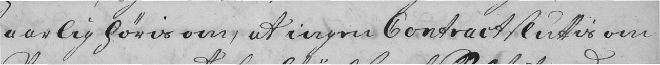aarlig høris om, at ingen Contract sluttis om
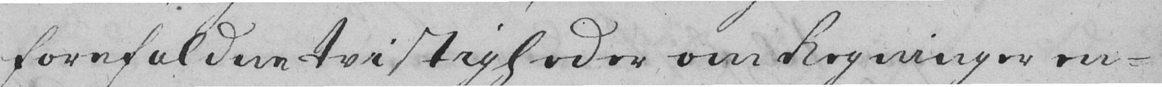forefalden tvistigheder om Regninger en-
